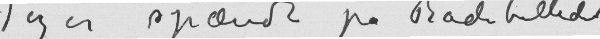Jeg er spændt på Badebilledet
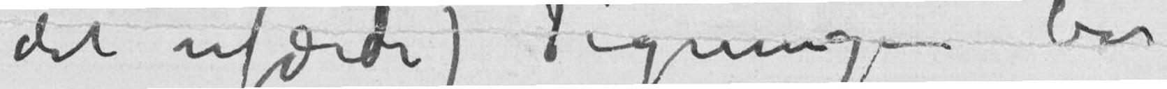det ufærdi) Tegningen bør
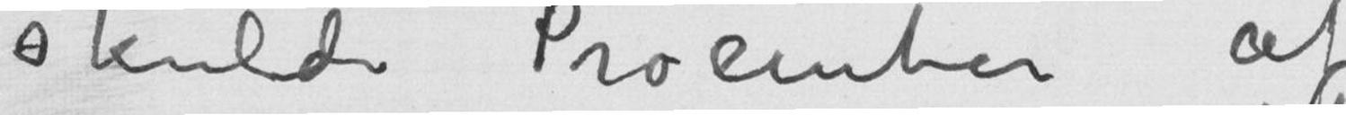skulde Procenter af
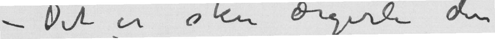– Det er sku ærgerli den
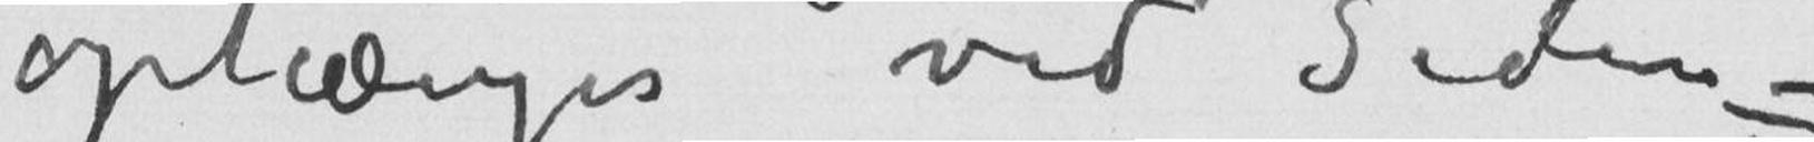ophænges ved Siden –
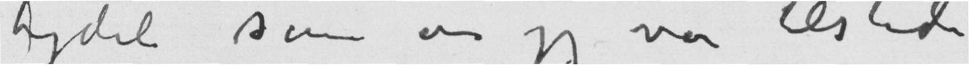tydeli som om jeg var tilstede
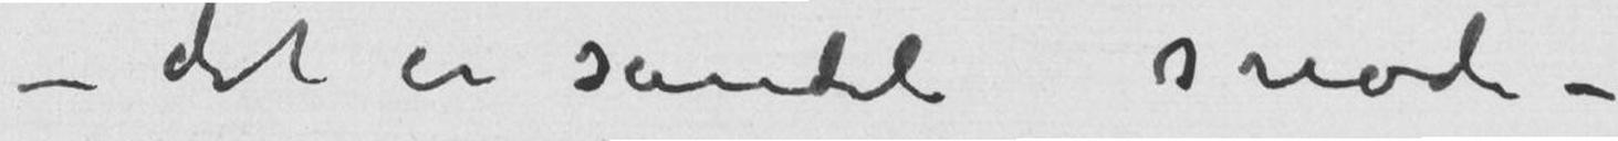– det er sandeli snodi –
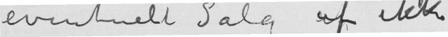eventuelt Salg af ikke
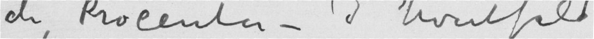de Procenter – I hvertfald
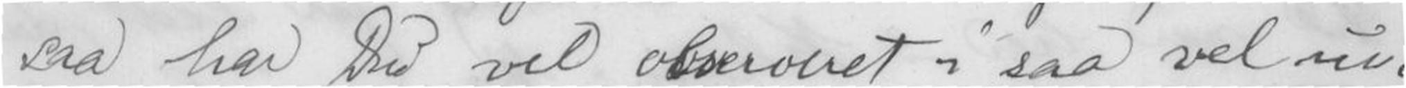saa har Du vel observeret i saa vel un-
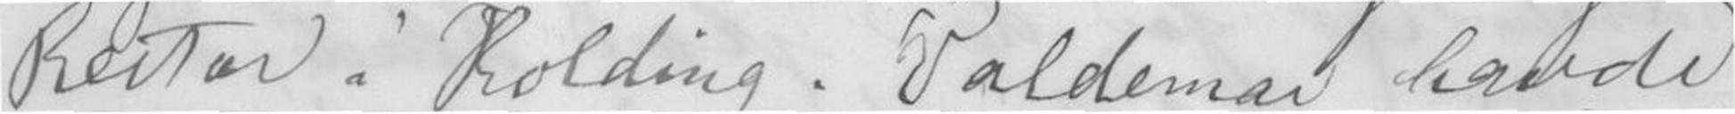Reitar i Kolding. Valdemar havde
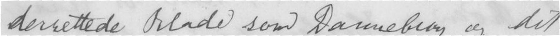derrettede Blade som Danneberg og det
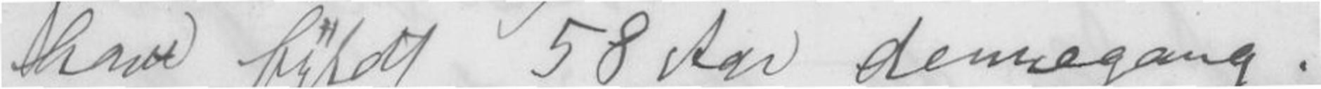have fyldt 58 Aar dennegang.
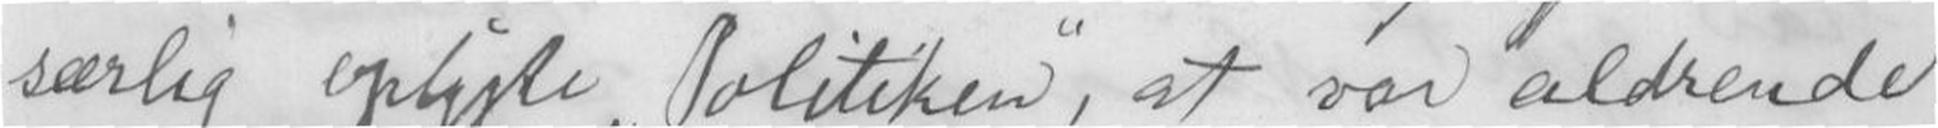særlig oplyste Politiken, at vor aldrende
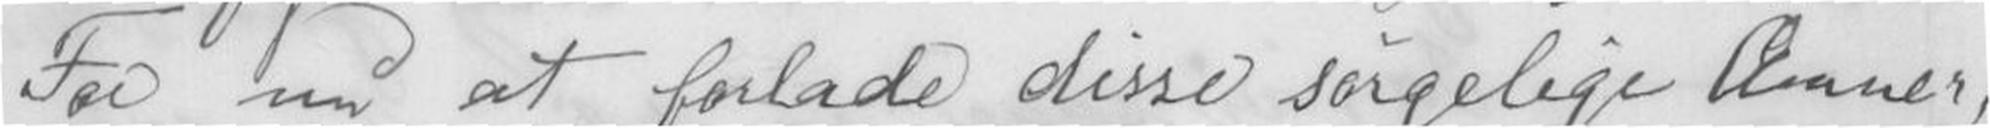For nu at forlade dissse sørgelige Æmner,
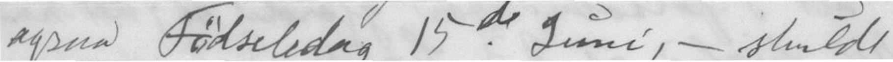ogsaa Fødselsdag 15de Juni, - skulde
